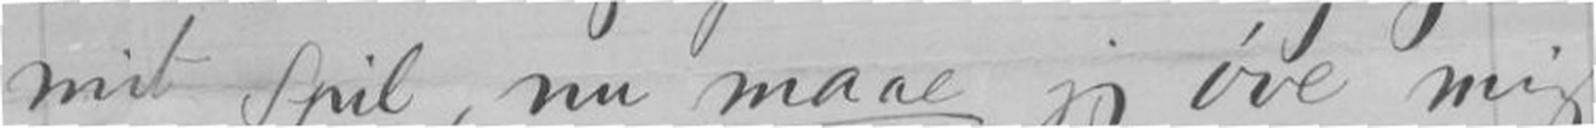mit Spil, nu maae jeg øve mig.
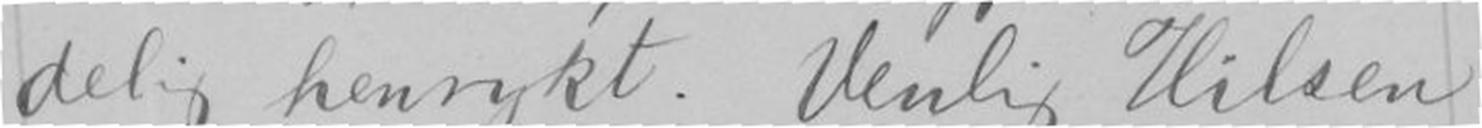delig henrykt. Venlig Hilsen
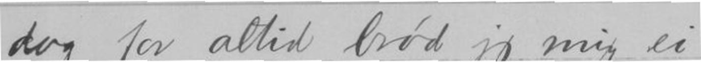dog for altid brød jeg mig ei
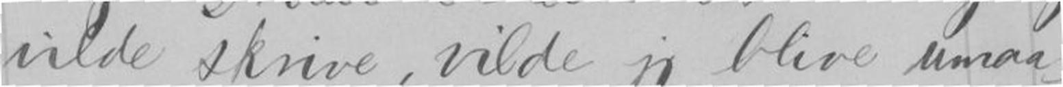vilde skrive, vilde jeg blive umaa-
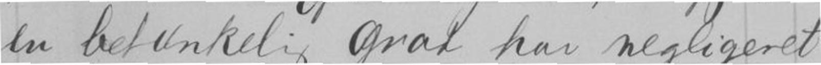en betænkelig Grad har negligeret
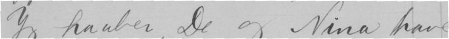Jeg haaber De og Nina have
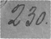230.
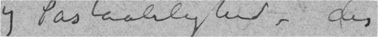og Påstaalelighed – der
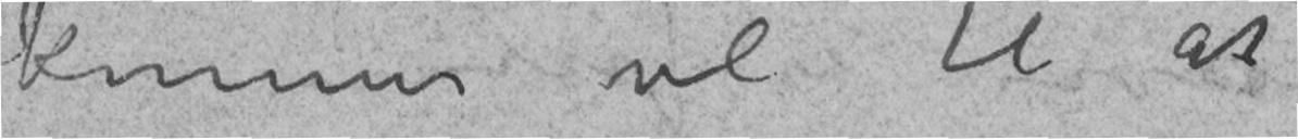kommer vel til at
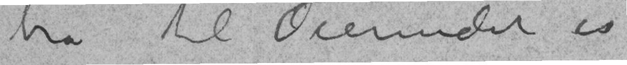bra til Ærendet er
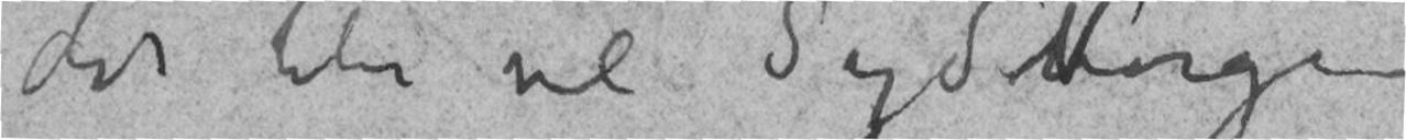det blir vel Syd Norge –
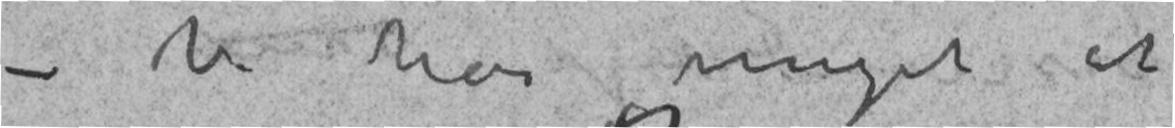– Vi har meget at
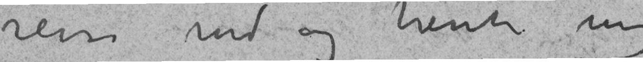reise ned og hente mig
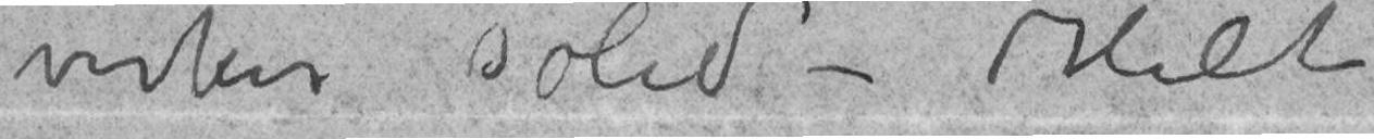virker solid – Helt
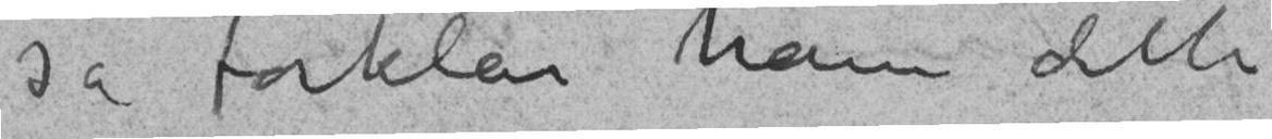så forklar ham dette
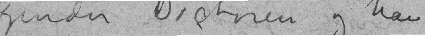kjender Doctoren og han
